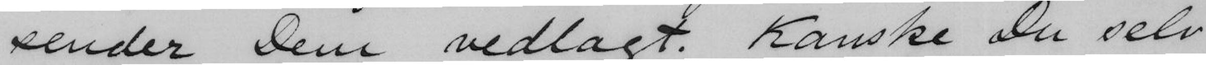sender Dem vedlagt. Kanske Du selv
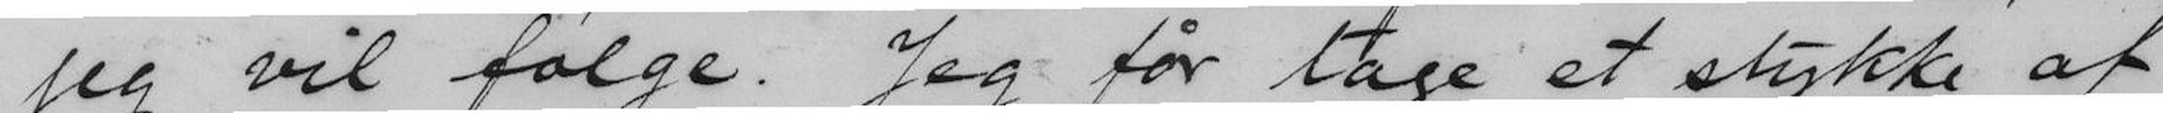jeg vil følge. Jeg får tage et stykke af
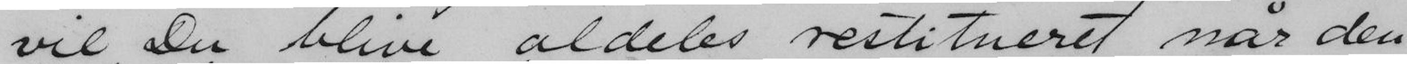vil Du blive aldeles restitueret når den
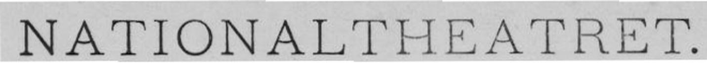NATIONALTHEATRET.
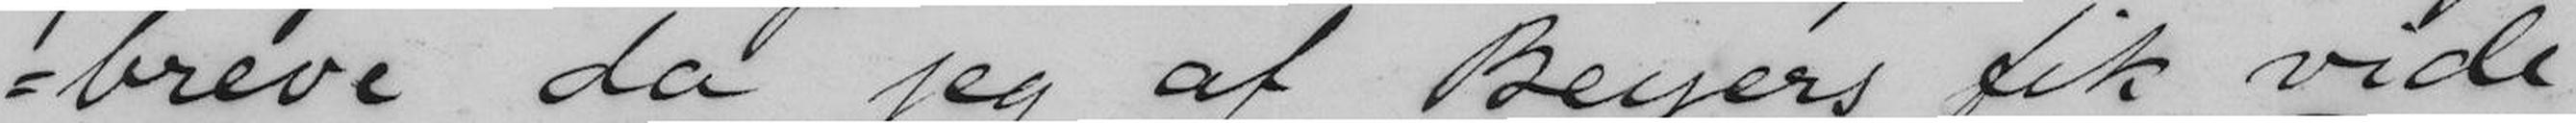breve da jeg af Beyers fik vide
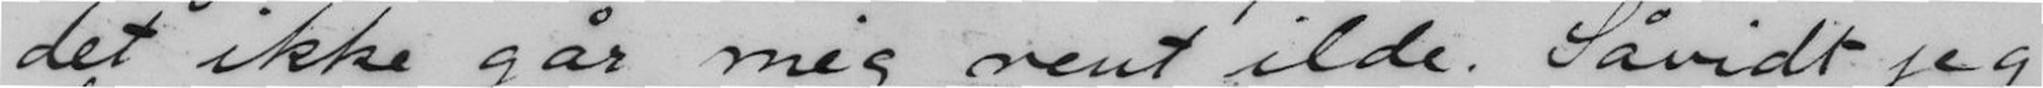det ikke går mig rent ilde. Såvidt jeg
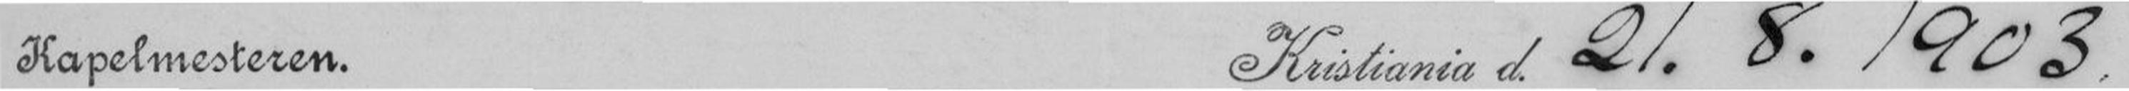Kapelmesteren.Kristiania d. 21. 8. 1903.
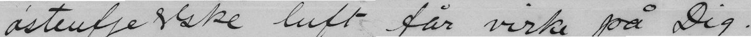østenfjeldske luft får virke på Dig.
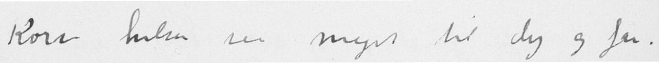Korre hilser saa meget til dig og far.
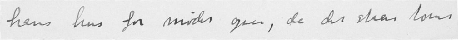hans hus for mødet ogsaa, da det staar tomt
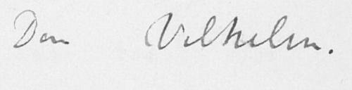Din Vilhelm.
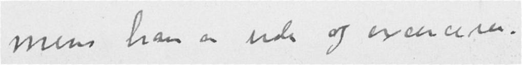mens han er ude og exerciser.
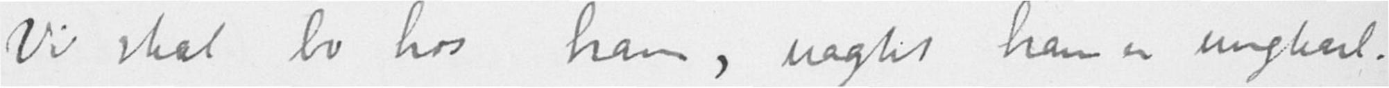Vi skal bo hos ham, uagtet han er ungkarl.
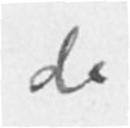da
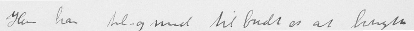Han har til og med tilbudt os at benytte
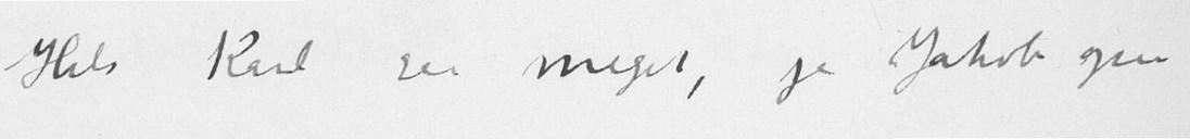Hils Karl saa meget, ja Jakob ogsaa
